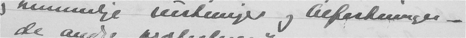og hemmelige rustninger og befestninger -
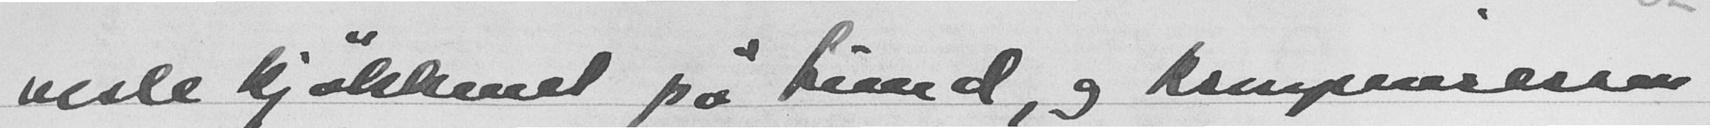viste kjøkkenet på hund, og kronprinsessen
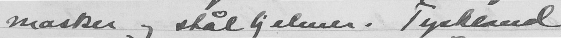masker og stålhjelmer. Tyskland
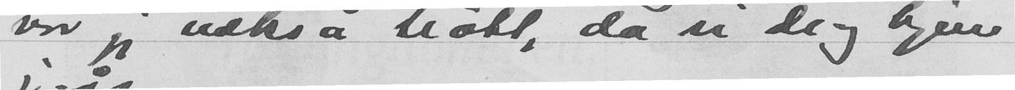var jeg nokså trøtt, da vi drog hjem
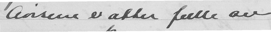Avisene er atter fulle av
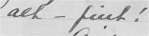alt - fint!
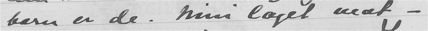barn er de. Nini laget mat -
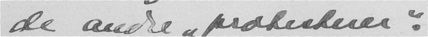de andre "protesterer".
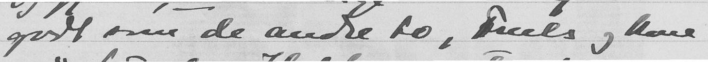godt som de andre to, Friels og kone
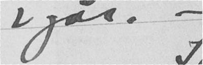igår. -
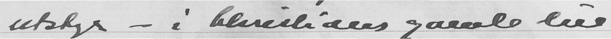utstyr - i Christians gamle lue
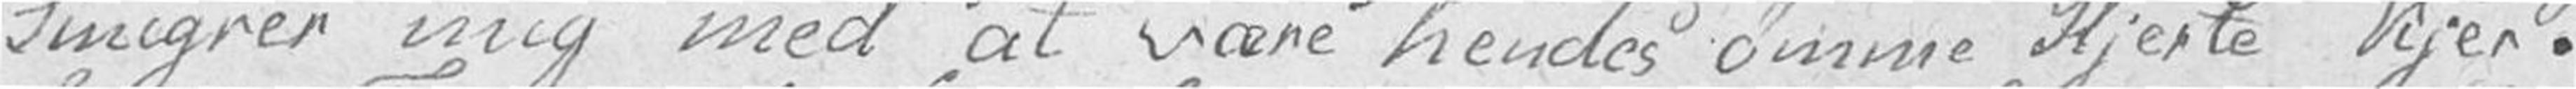Smigrer mig med at være hendes ømme Hjerte kjer.
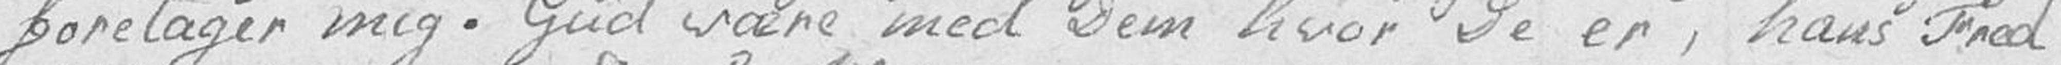foretager mig. Gud være med Dem hvor De er, hans Fred
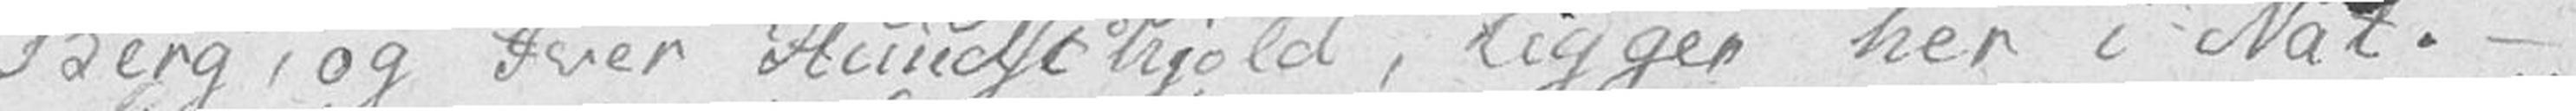Berg, og Iver Hundschjold, ligger her i Nat. –
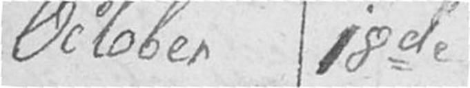October 18de
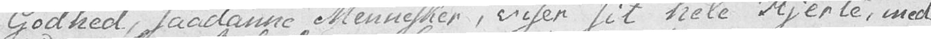Godhed, saadanne Mennesker, viser sit hele Hjerte, med
